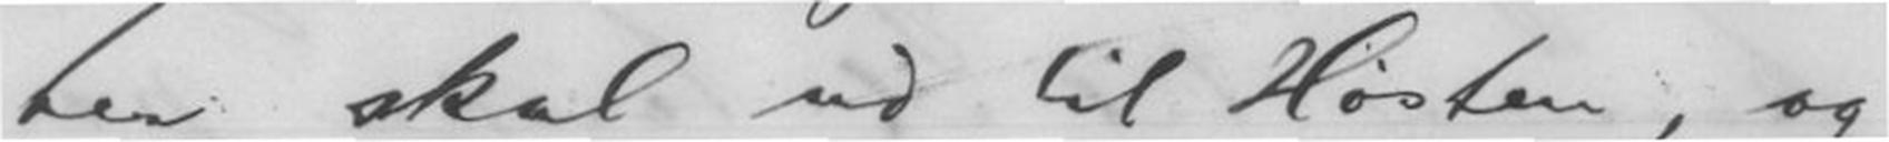ter skal ud til Høsten,
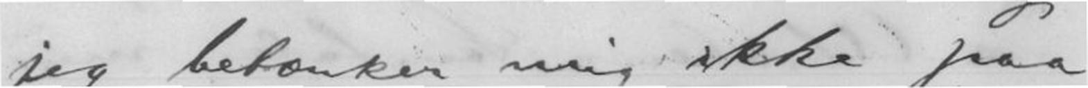og jeg betænker mig ikke paa
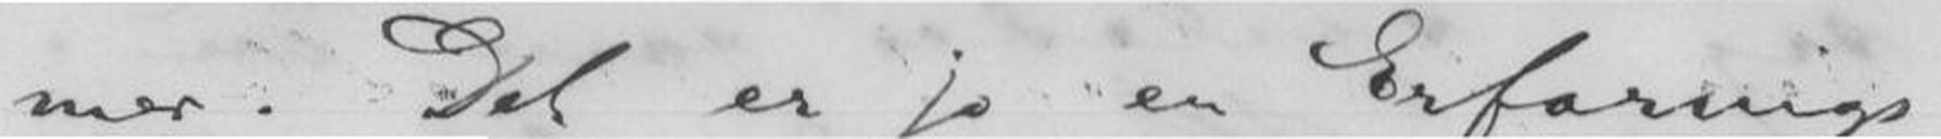mer. Det er jo en Erfarings-
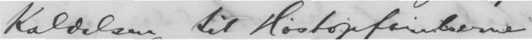Kaldelsen til Høstopførelserne
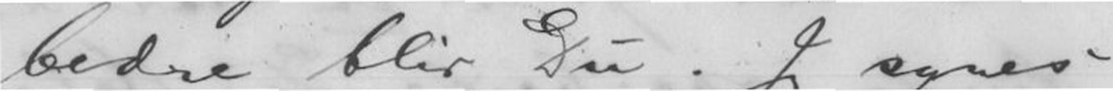bedre blir Du. Jeg synes
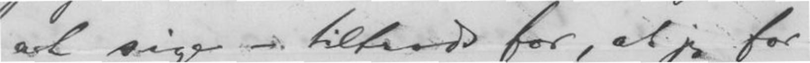at sige - tiltrods for, at jeg for-
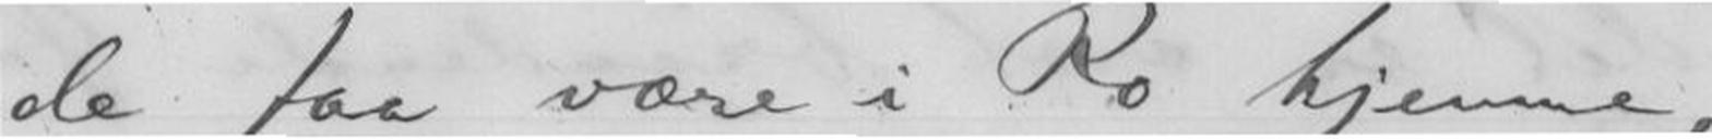de faa være i Ro hjemme,
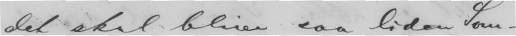det skal blive saa liden Som-
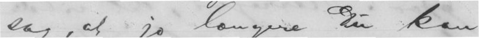sag, at jo længere Du kan
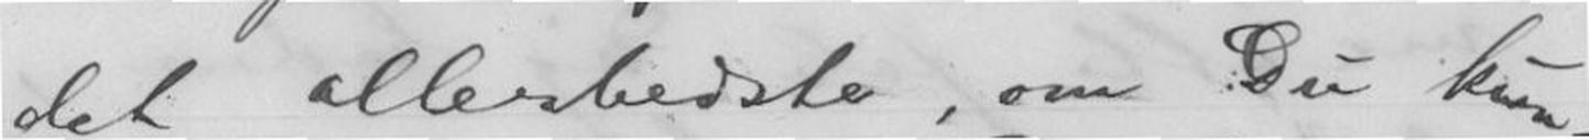det allerbedste, om Du kun-
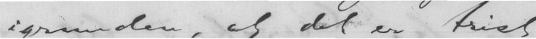igrunden, at det er trist
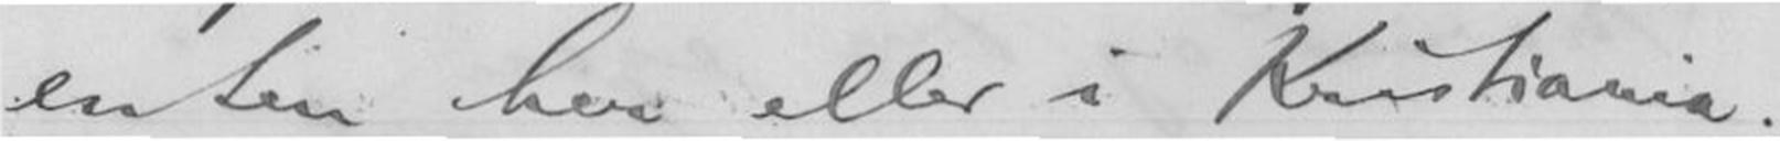enten her eller i Kristiania.
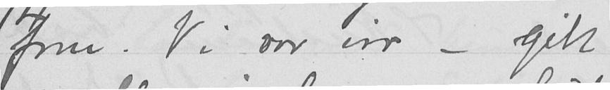form. Vi var vør - gik
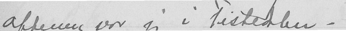aftenen var jeg i Tistedalen.
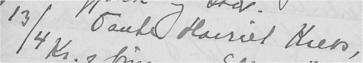13/4 Tante Harriet Kiers,
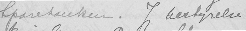Sparebanken. Jeg bestyrelse
